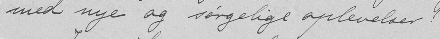med nye og sørgelige oplevelser!
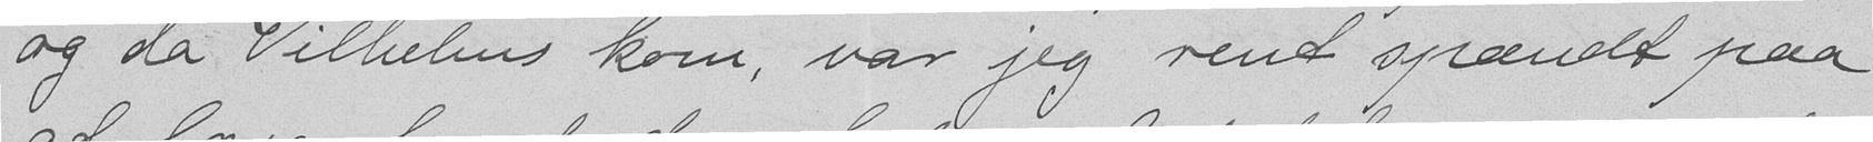og da Vilhelms kom, var jeg rent spændt paa
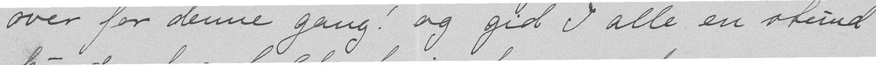over for denne gang! og gid I alle en stund
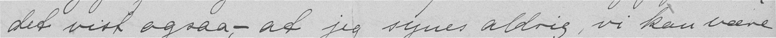det vist ogsaa, - at jeg synes aldrig, vi kan være
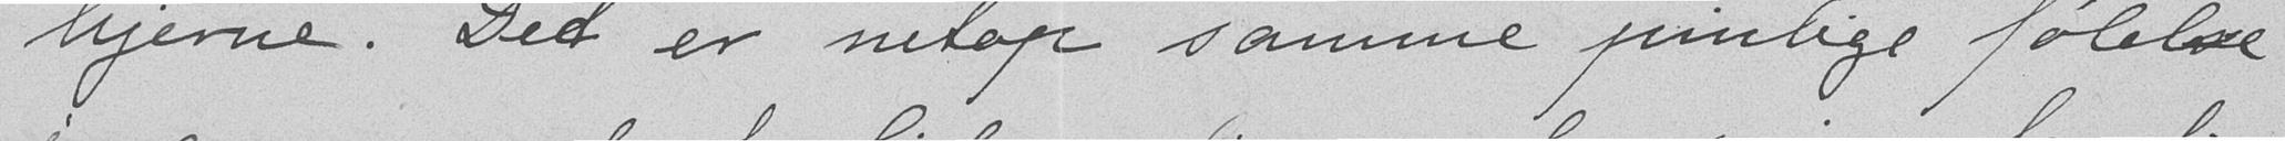hjerne. Det er netop samme pinlige følelse
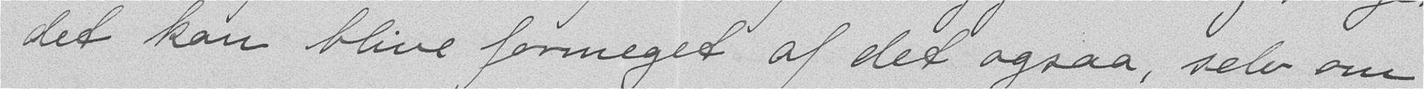det kan blive formeget af det ogsaa, selv om
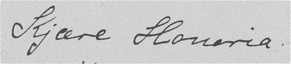Kjære Honoria!
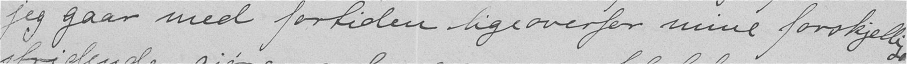jeg gaar med fortiden ligeoverfor mine forskjellige
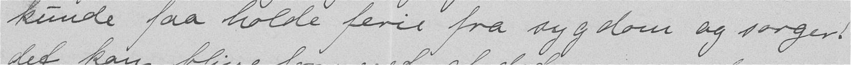kunde faa holde ferie fra sygdom og sorger!
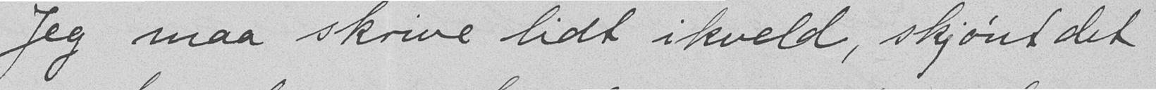Jeg maa skrive lidt ikveld, skjønt det
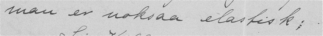man er noksaa elastisk.
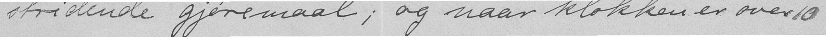stridende gjøremaal; og naar klokken er over 10
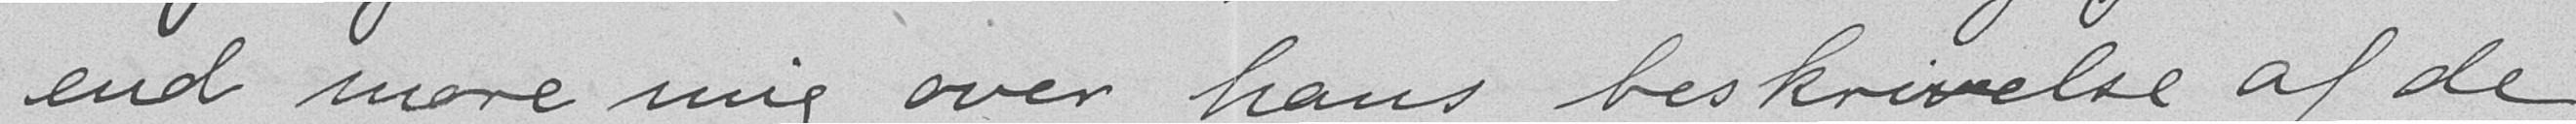end more mig over hans beskrivelse af de
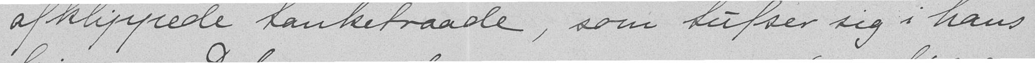afklippede tanketraade, som tufser sig i hans
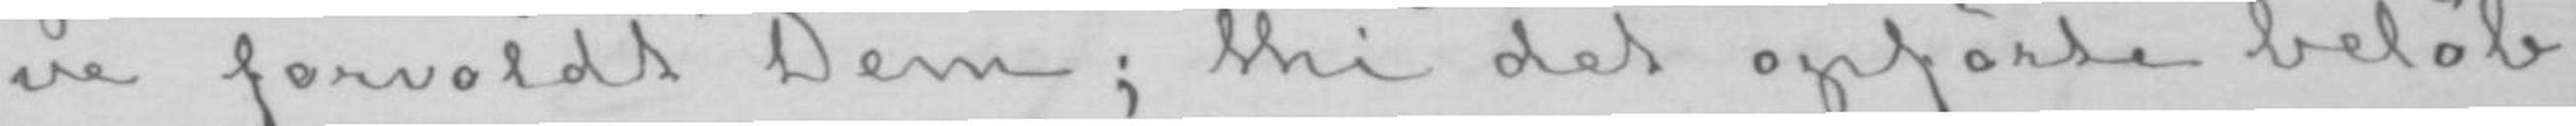ve forvoldt Dem; thi det opførte beløb
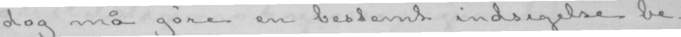jeg dog må gøre en bestemt indsigelse be-
